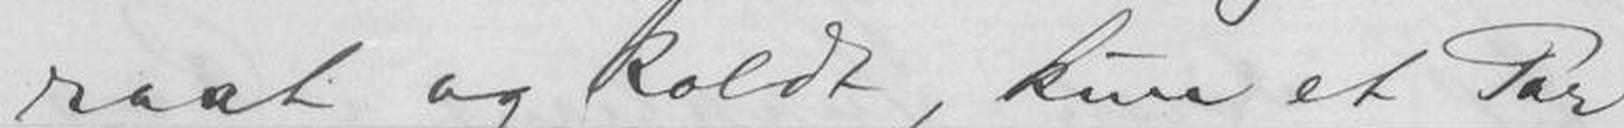raat og koldt, kun et Par
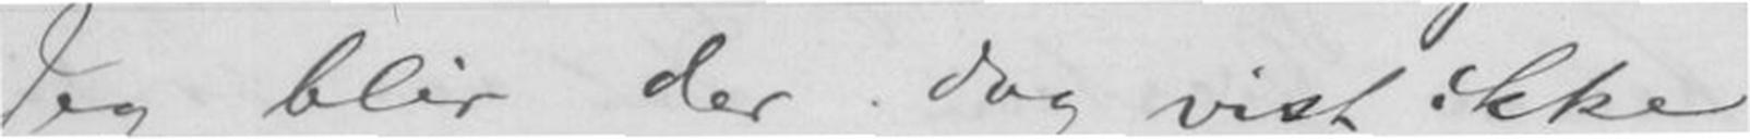Jeg blir der dog vist ikke
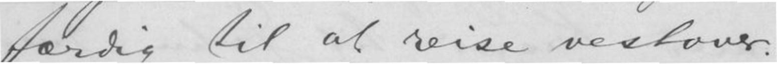færdig til at reise vestover.
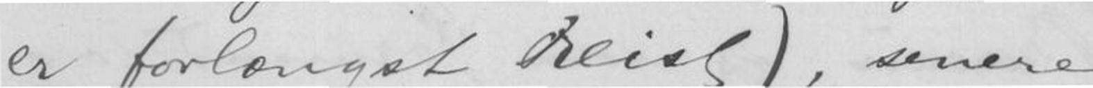er forlængst reist), senere
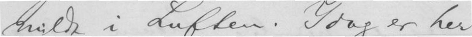mildt i Luften. Idag er her
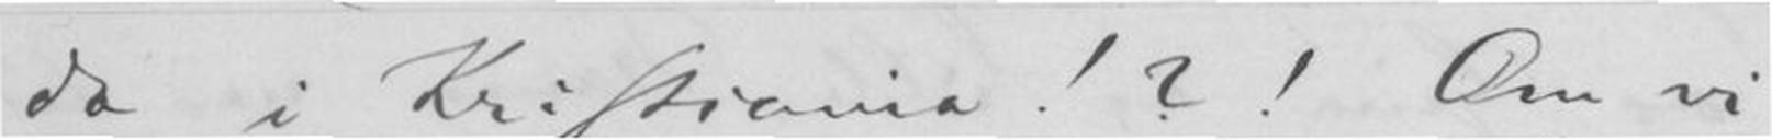da i Kristiania!?! Om vi
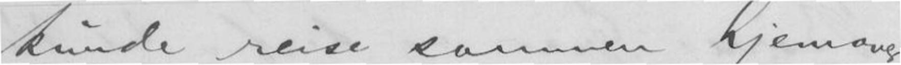kunde reise sammen hjemover!
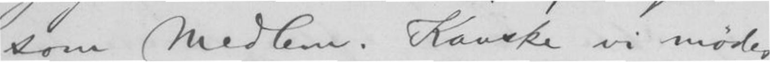som Medlem. Kanske vi mødes
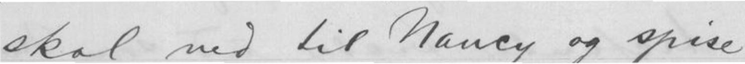skal ned til Nancy og spise
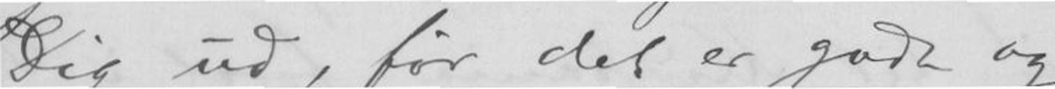Dig ud, før det er godt og
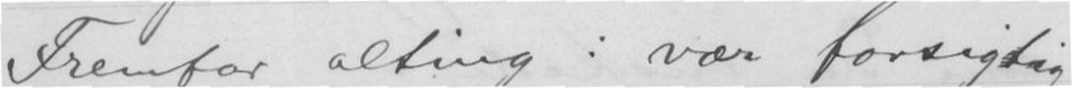Fremfor alting: vær forsigtig,
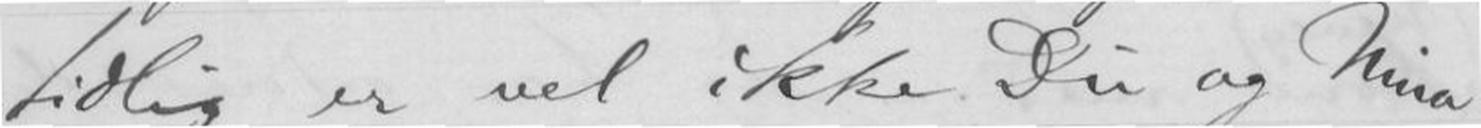tidlig er vel ikke Du og Nina
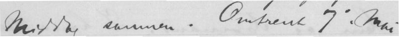Middag sammen. Omtrent 7de Mai
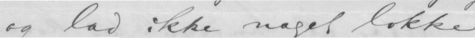og lad ikke noget lokke
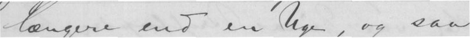længere end en Uge, og saa
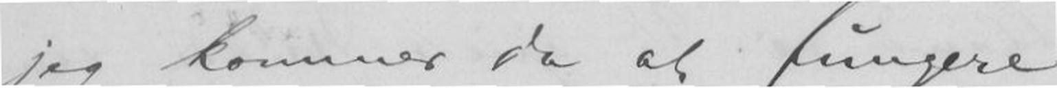jeg kommer da at fungere
